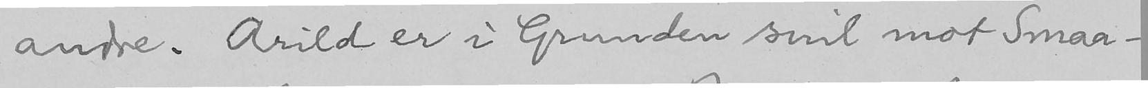andre. Arild er i Grunden snil mot Smaa-
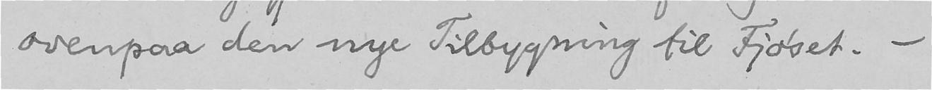ovenpaa den nye Tilbygning til Fjøset. -
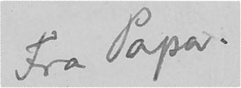Fra Papa.
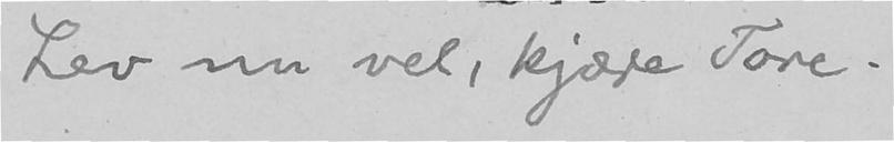Lev nu vel, kjære Tore.
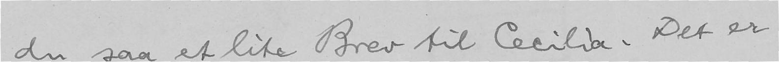du saa et lite Brev til Cecilia. Det er
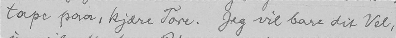tape paa, kjære Tore. Jeg vil bare dit Vel,
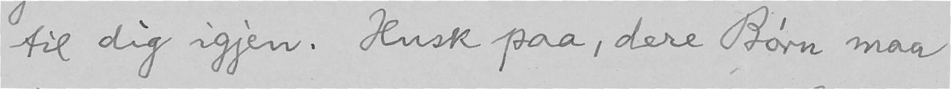til dig igjen. Husk paa, dere Børn maa
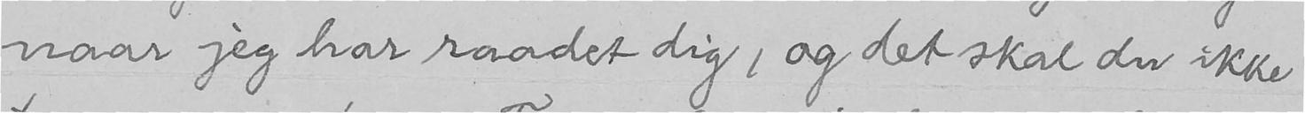naar jeg har raadet dig, og det skal du ikke
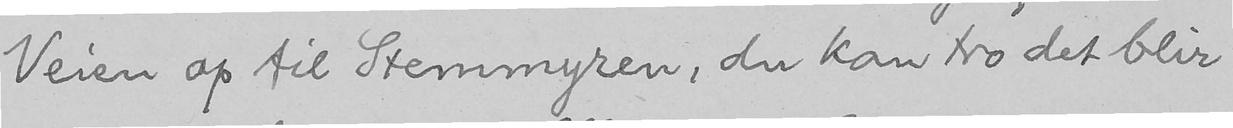Veien op til Stemmyren, du kan tro det blir
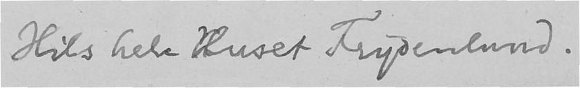Hils hele Huset Frydenlund.
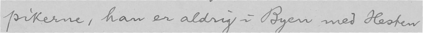pikerne, han er aldrig i Byen med Hesten
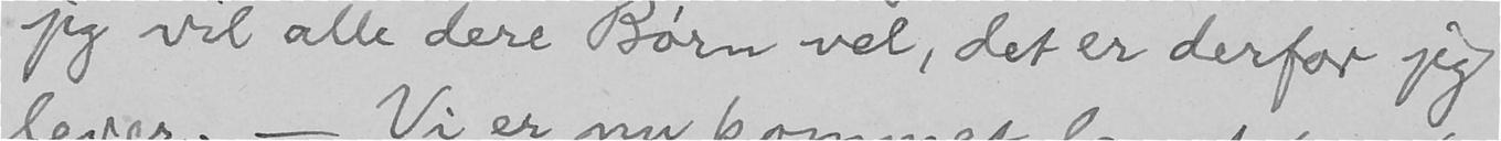jeg vil alle dere Børn vel, det er derfor jeg
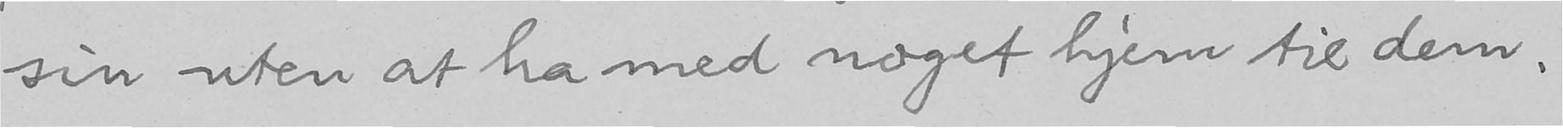sin uten at ha med noget hjem til dem.
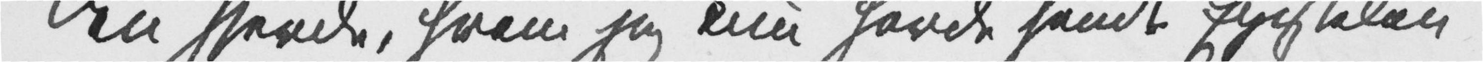Den fjerde, hvem jeg kun havde sendt Epistelen
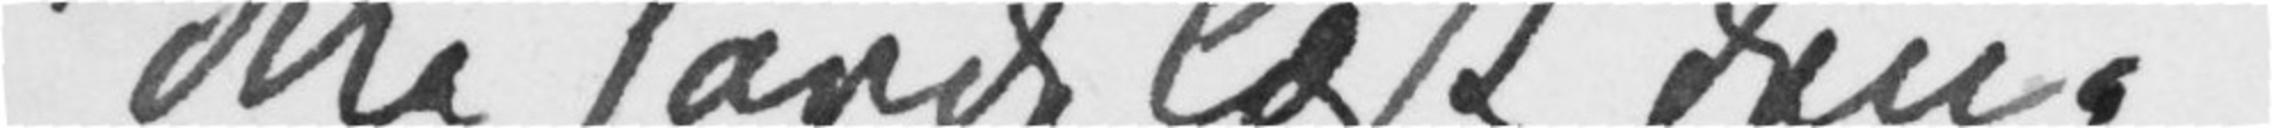«ikke havde læst den».
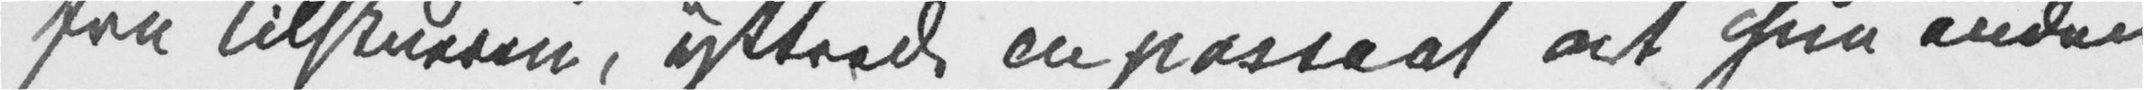fra «Tilskueren», yttrede en passant at hun endnu
